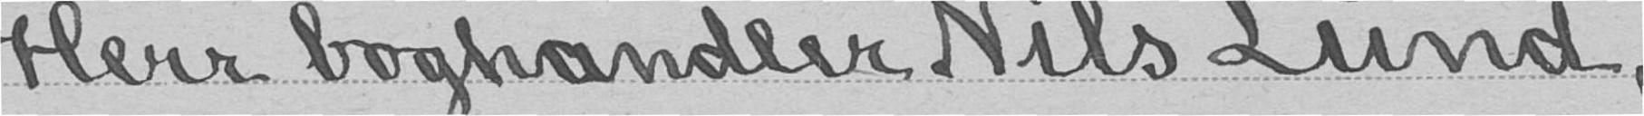Herr boghandler Nils Lund,
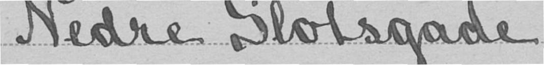Nedre Slotsgade
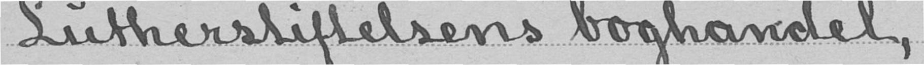Lutherstiftelsens boghandel.
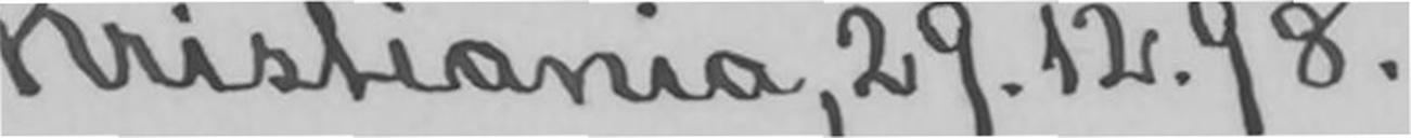Kristiania, 29.12.98.
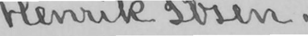Henrik Ibsen.
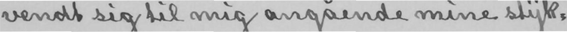vendt sig til mig angående mine styk-
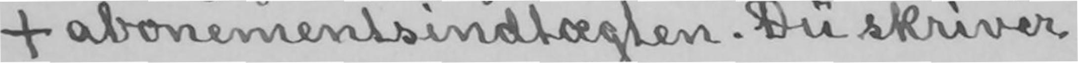+ abonementsindtægten. Du skriver
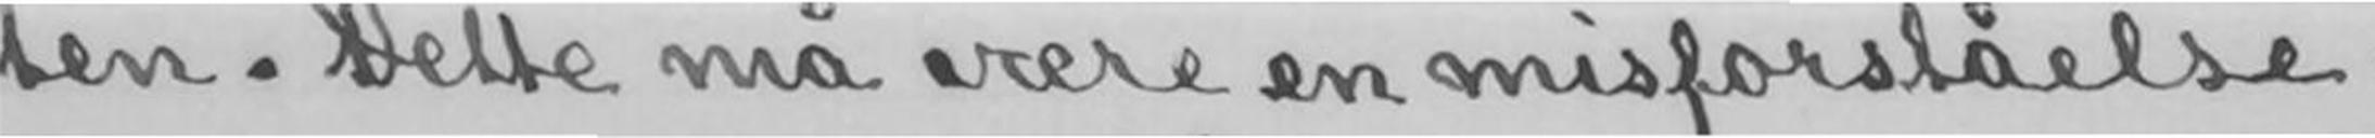ten. Dette må være en misforståelse.
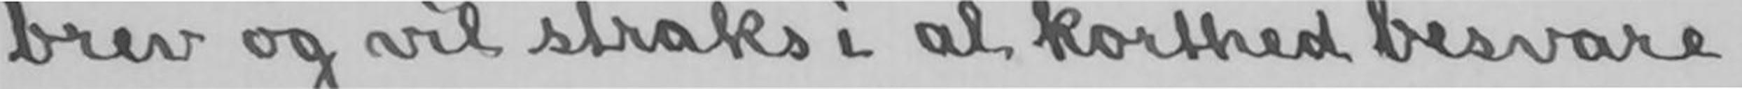brev og vil straks i al korthed besvare
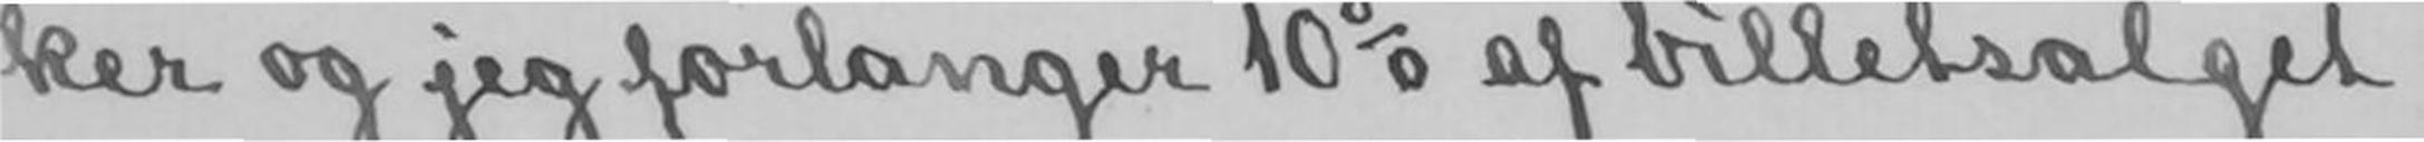ker og jeg forlanger 10% af billetsalget
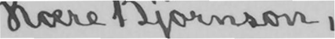Kære Bjørnson,
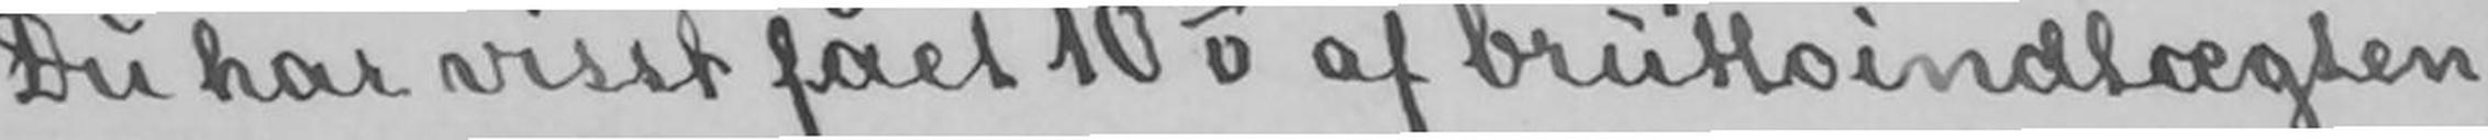Du har visst fået 10% af bruttoindtægten
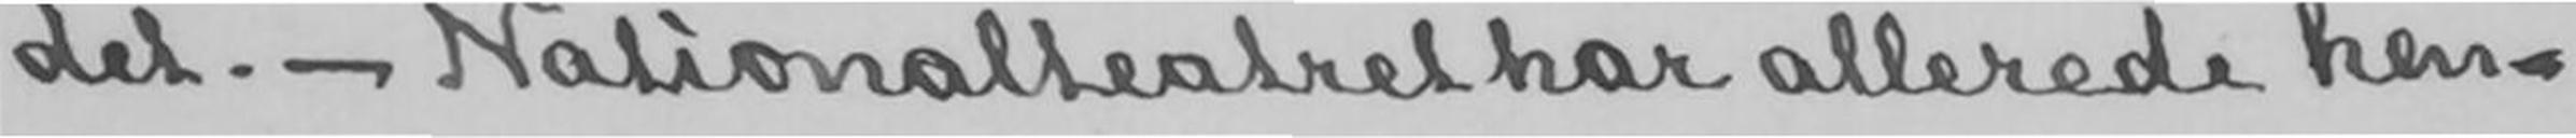det.- Nationalteatret har allerede hen-
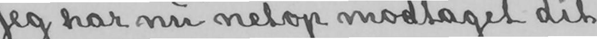Jeg har nu netop modtaget dit
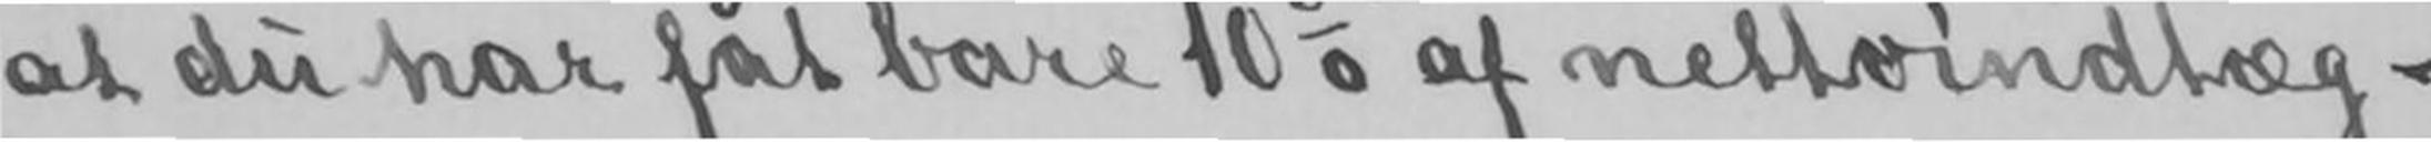at du har fåt bare 10%  af nettoindtæg-
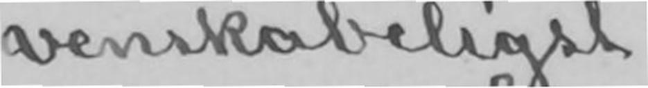venskabeligst
